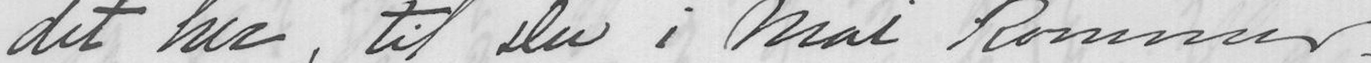det her, til Du i Mai kommer.
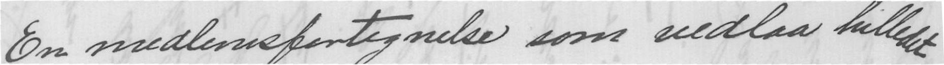En medlemsfortegnelse som vedlaa billedet
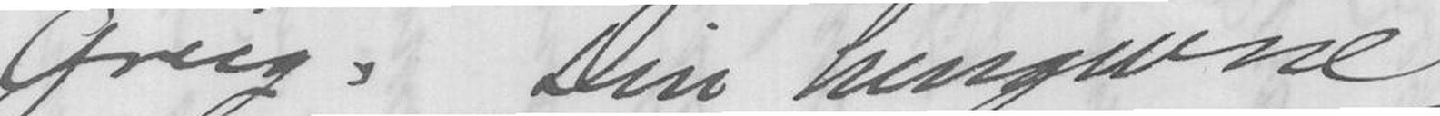Grieg. Din hengivne
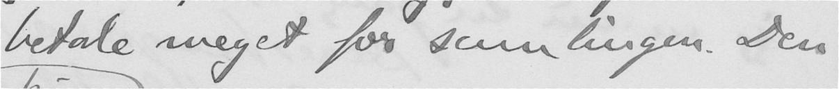betale meget for samlingen. Den
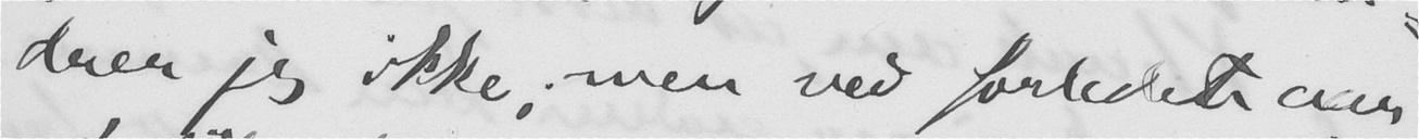drer jeg ikke, men ved forleden aar
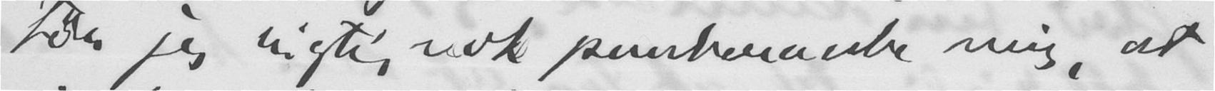tør jeg rigtig nok paaberaabe mig, at
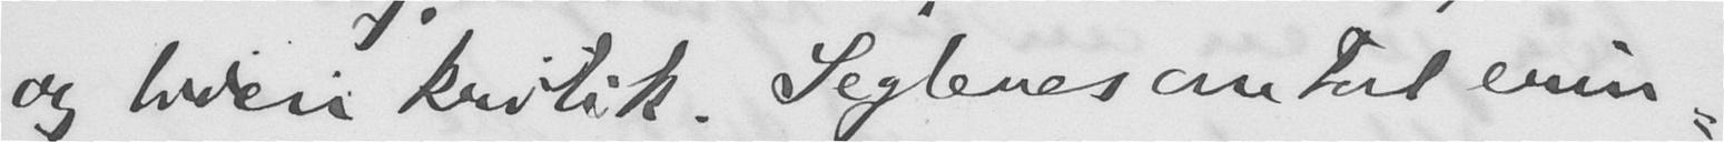og liden kritik. Seglenes antal erin-
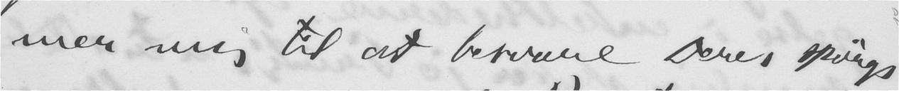mer mig til at besvare Deres spørgs-
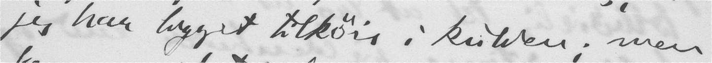jeg har ligget tilkøis i kulden; men
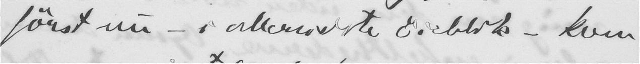først nu – i allersidste øieblik – kom-
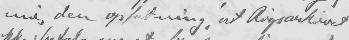mig den opfatning, at Rigsarkivet
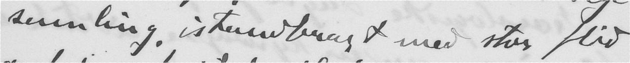samling, istandbragt med stor flid
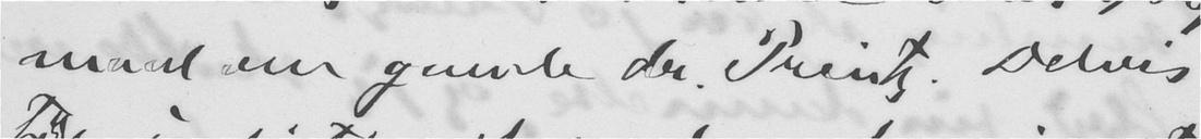maal om gamle dr. Printz. Delvis
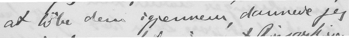at løbe dem igjennem, dannede jeg
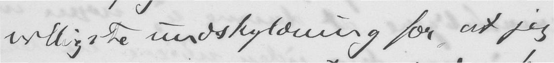villigste undskyldning for, at jeg
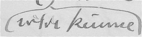vilde kunne
